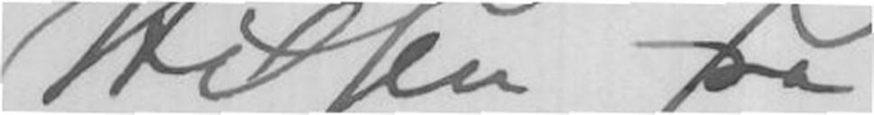Hilsen fra
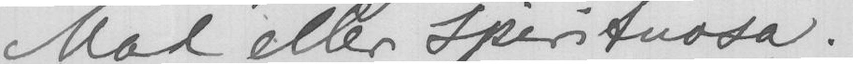Mad eller Spirituosa. -
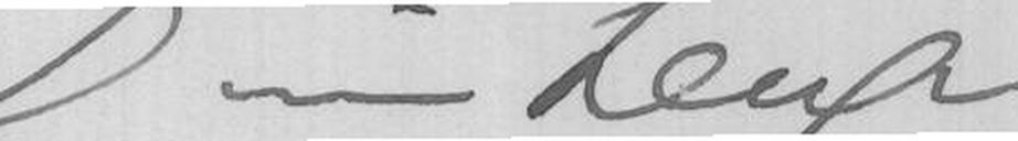Din hengivne
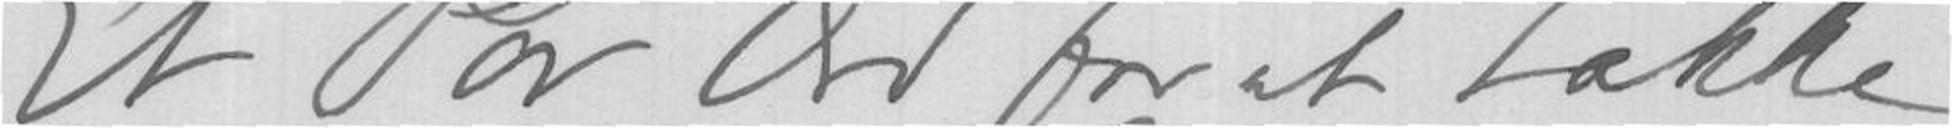Et Par Ord for at takke
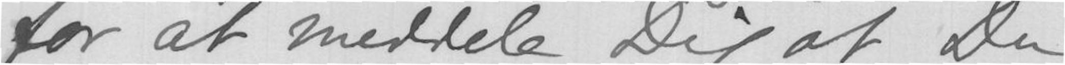for at meddele Dig at Du
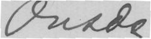Onsdag
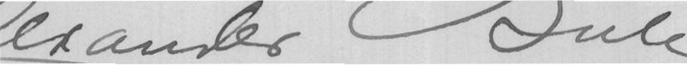Alexander Bull
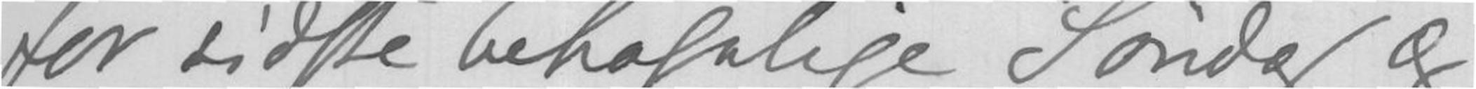for sidste behagelige Søndag og -
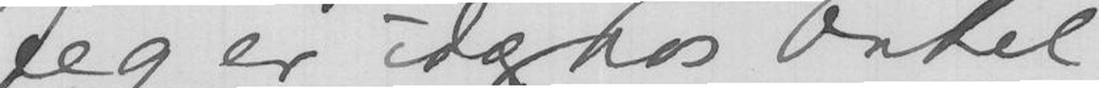Jeg er idag hos Onkel
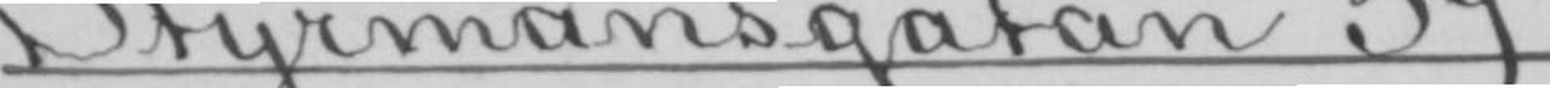Styrmansgatan 39.
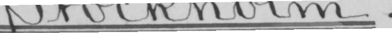Stockholm.
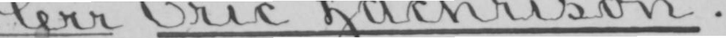Herr Eric Zachrison.
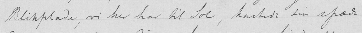Blikplade vi her har til Sol, kastede sin spæde
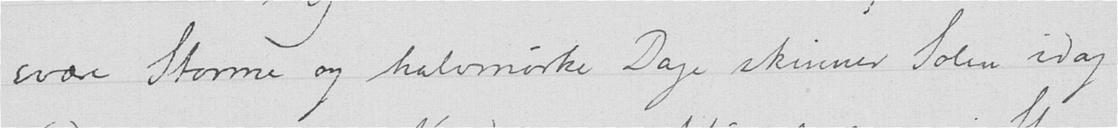svære Storme og halvmørke Dage skinner Solen idag
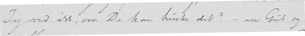Jeg ved ikke, om De kan huske det? - en Gut og
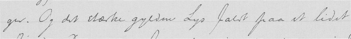ger. Og det stærke gyldne Lys faldt paa et lidet
